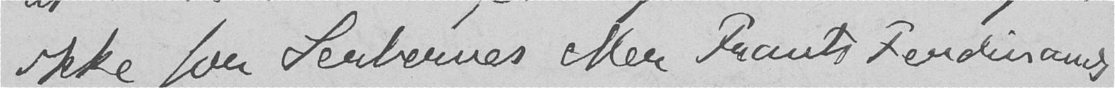ikke for Serbernes eller Frants Ferdinands
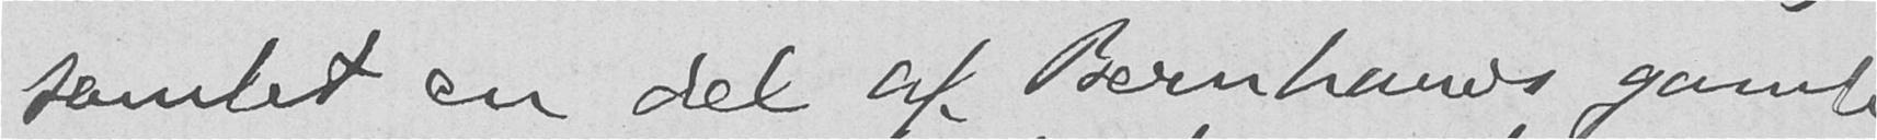samlet en del af Bernhards gamle
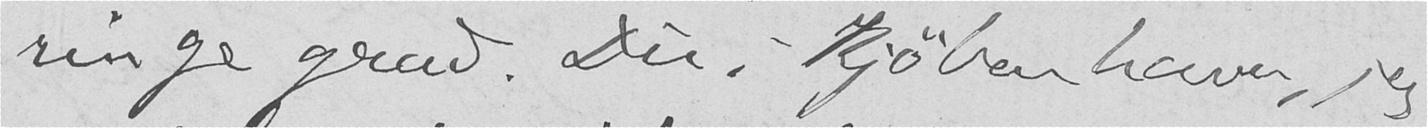ringe grad. Du i Kjøbenhavn, jeg
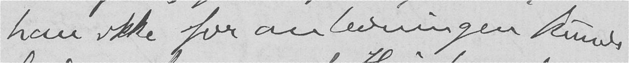han ikke for anledningen kunde
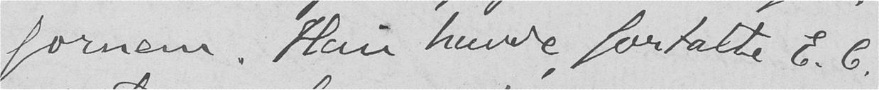fornem. Han havde, fortalte E. C.
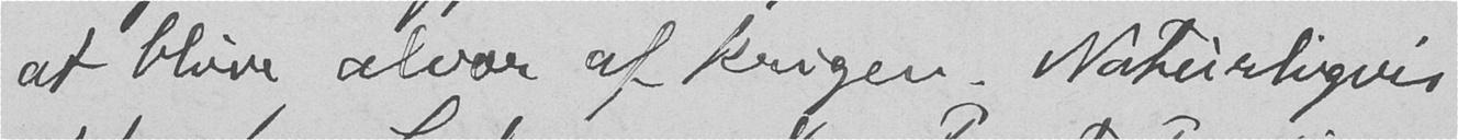at blive alvor af krigen. Naturligvis
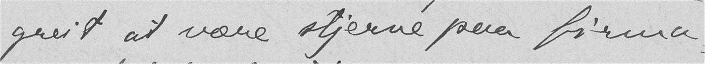greit at være stjerne paa firma-
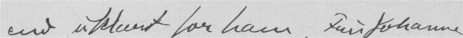end uklart for ham. Fru Johanne
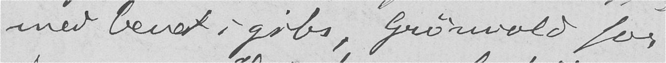med benet i gibs, Grønvold for
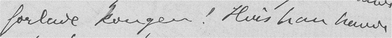forlade kongen! Hvis han havde
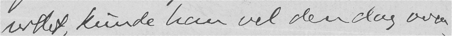villet, kunde han vel den dag over-
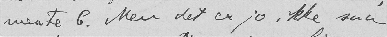mente C. Men det er jo ikke saa
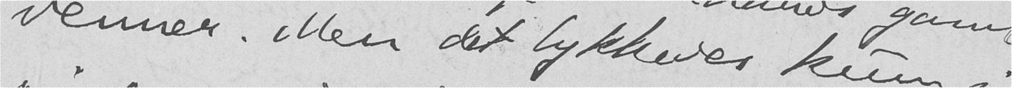venner. Men det lykkedes kun i
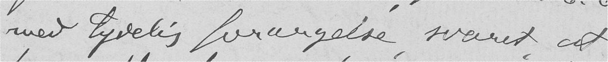med tydelig forargelse, svaret, at
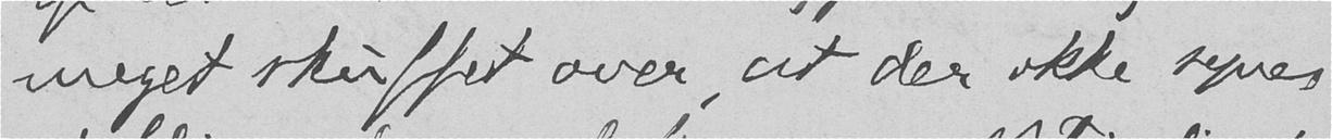meget skuffet over, at der ikke synes
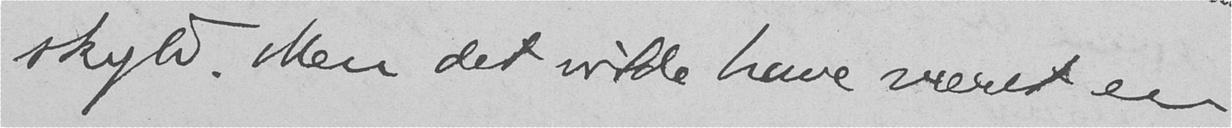skyld. Men det vilde have været en
